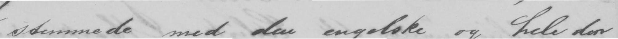stemmede med den engelske og hele den
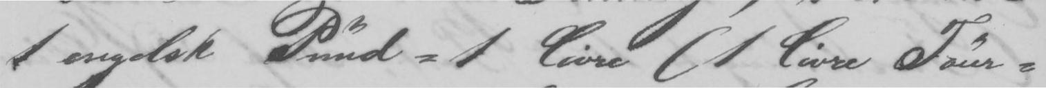1 engelsk Pund = 1 livre (1 livre Tour-
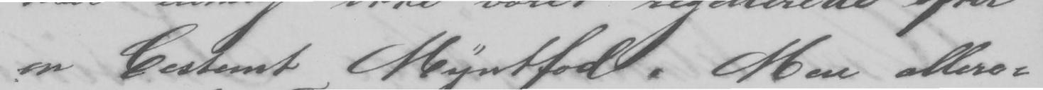en bestemt Myntfod. Men allere-
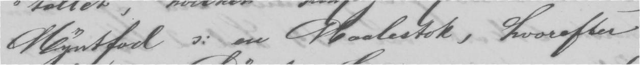Myntfod ɔ: en Maalestok, hvorefter
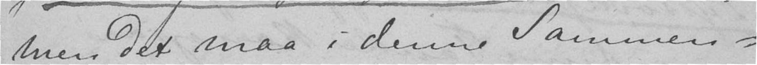men det maa i denne Sammen-
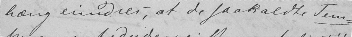hæng erindres, at de saakaldte Tem-
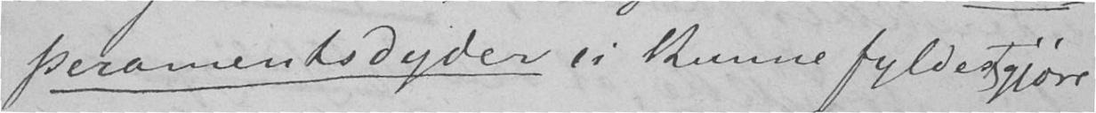peramentsdyder ei kunne fyldestgjøre
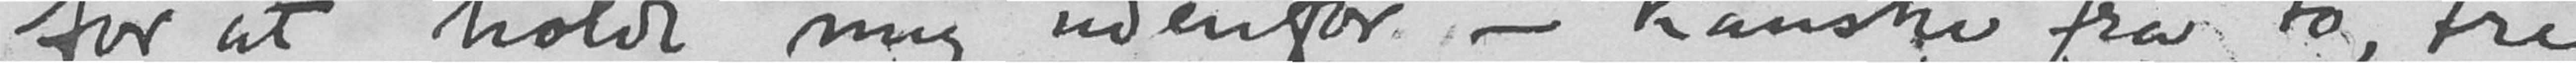for at holde mig udenfor - kanske fra to, tre
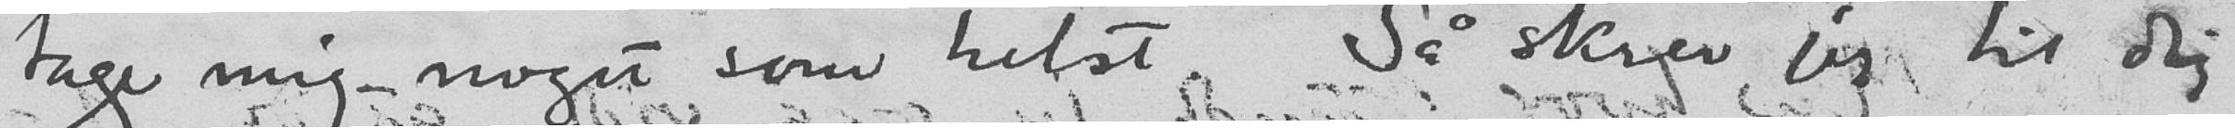tage mig noget som helst. Så skrev jeg til dig.
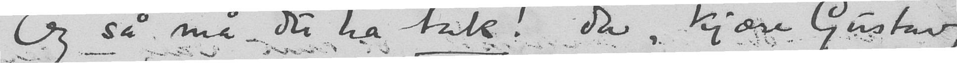Og så må du ha tak! da, kjære Gustav,
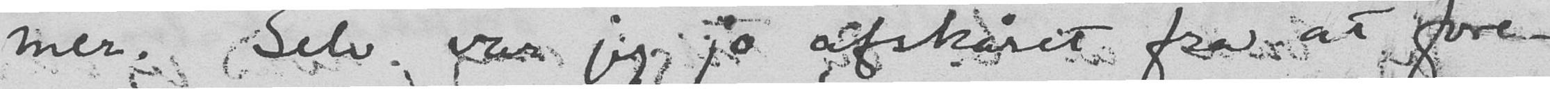mer. Selv var jeg jo afskåret fra at fore-
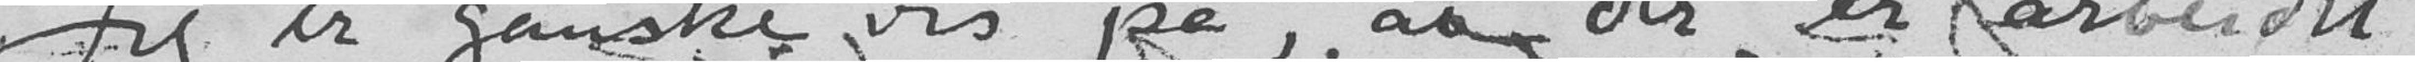Jeg er ganske vis på, at der er arbeidet
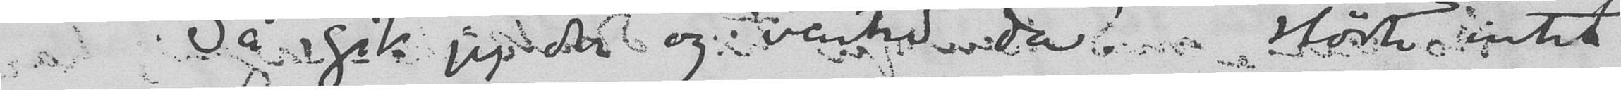Så gik jeg der og vented da! Hørte intet
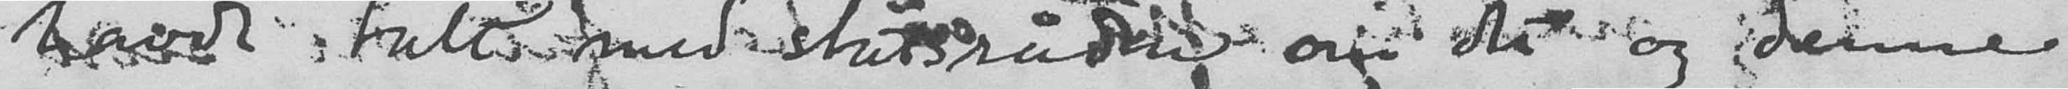havde talt med statsråden om det og denne
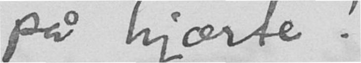på hjærte!
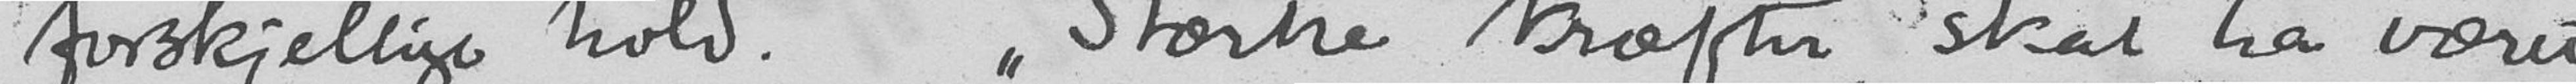forskjellige hold. "Stærke kræfter skal ha været
